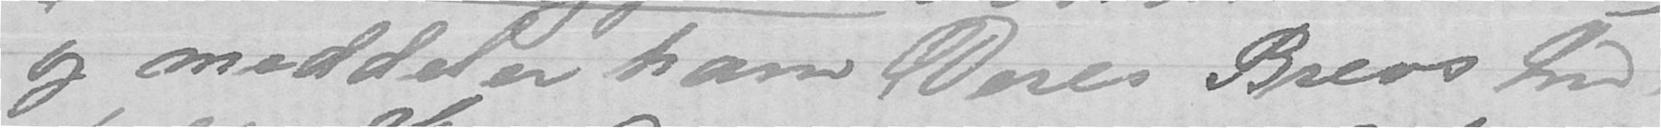og meddeler ham Deres Brevs Ind-
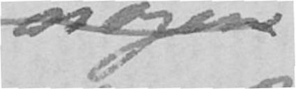nogen
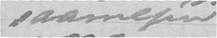saamegen
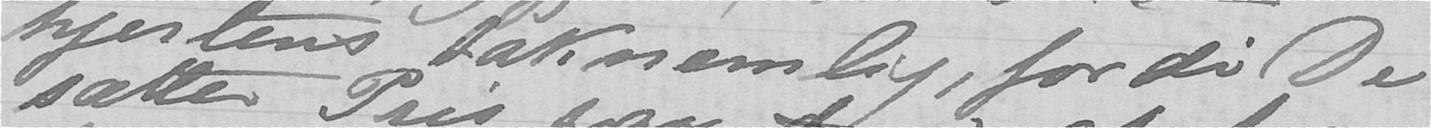hjertens taknemlig, fordi De
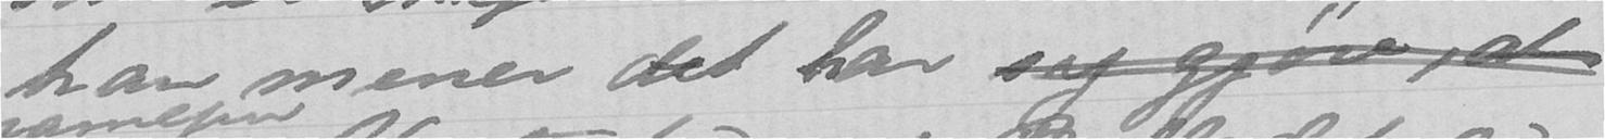han mener det har sig gjøre, at
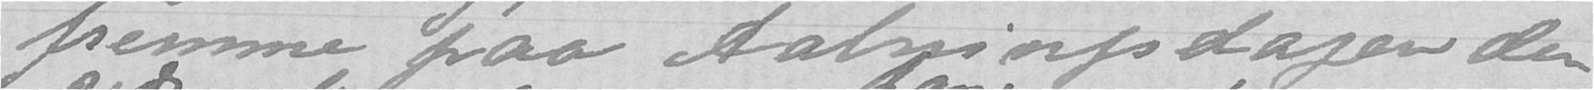fremme paa Aabningsdagen den
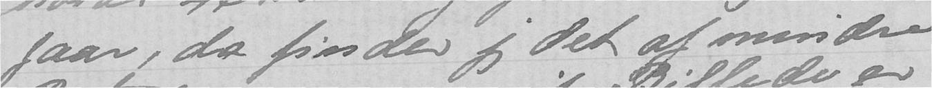gaar, da finder jeg det af mindre
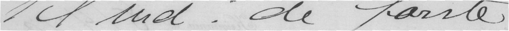Til ind i de første
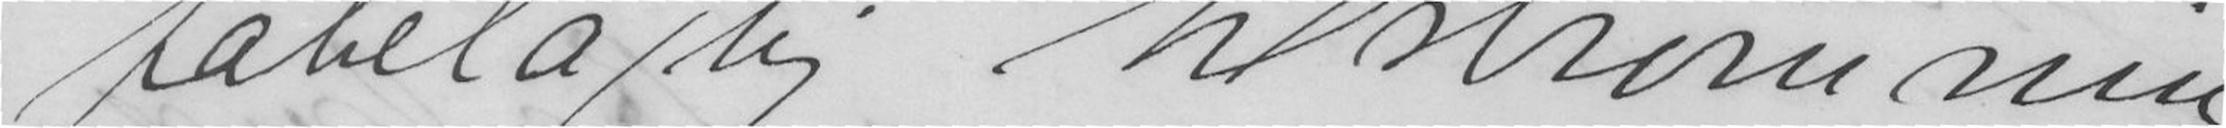fabelagtig tilstrømning.
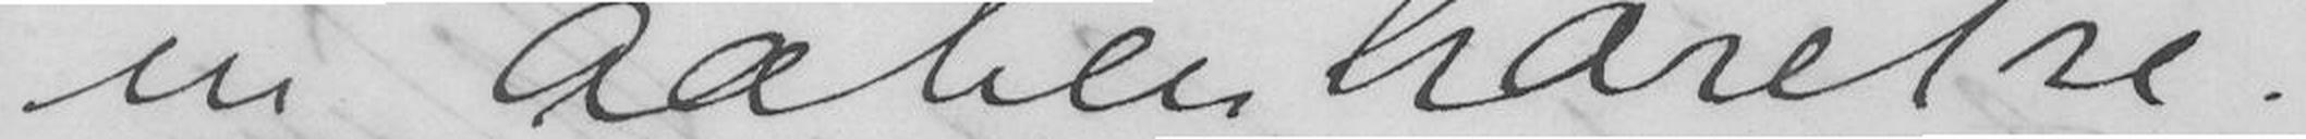en aabenbarelse!
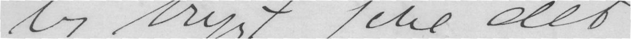vi trygt give det.
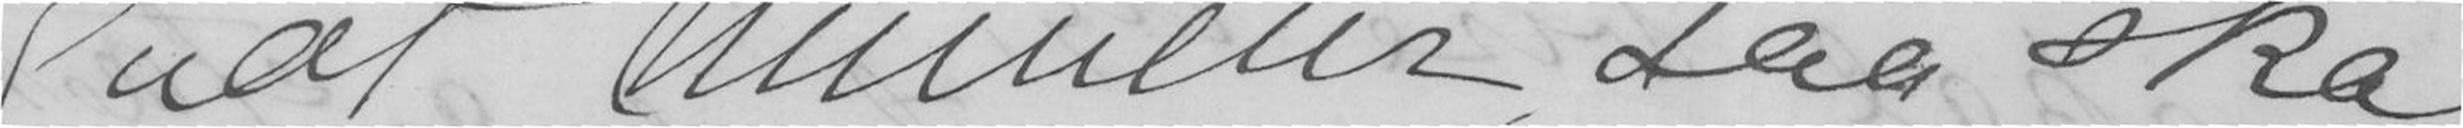gaat humeur, saa skal
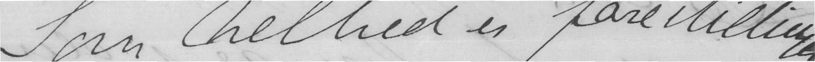Som helhed er forestillingen
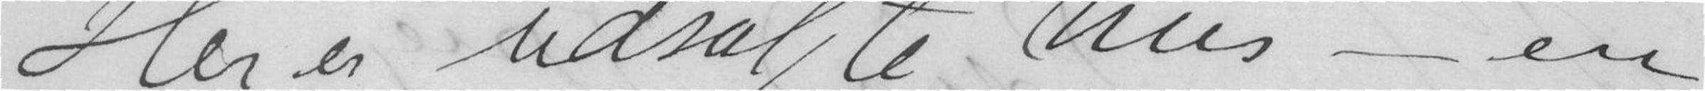Her er udsolgte hus - en
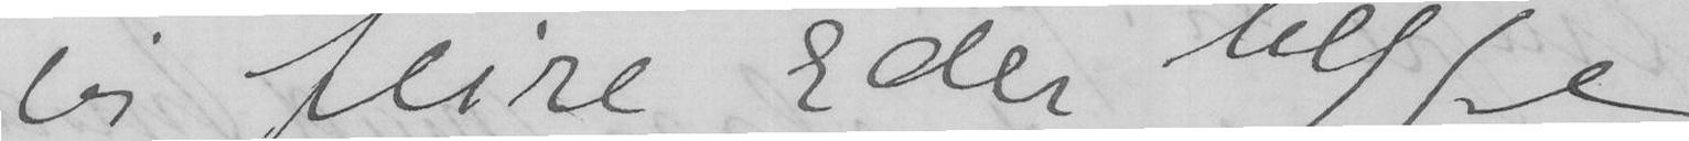vi feire Eder begge
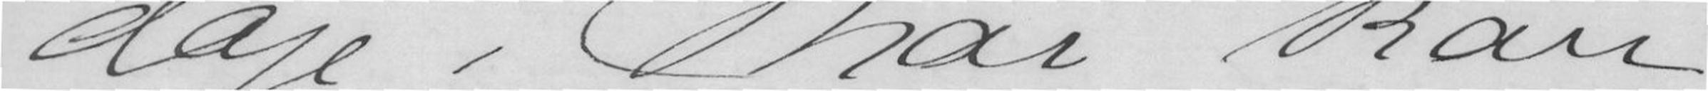dage i Mai kan
